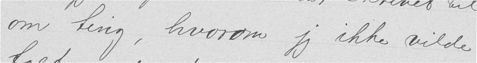om ting, hvorom jeg ikke vilde
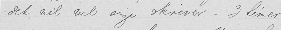– det vil vel sige skriver – 3 timer
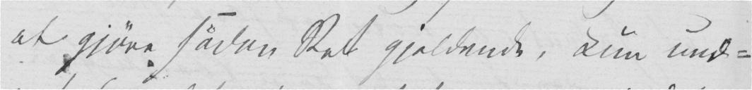at gjøre sådan Ret gjeldende, kun und-
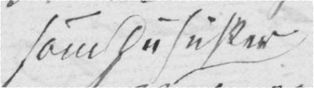som Du husker,
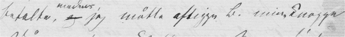betalte, og jeg måtte aftigge B. mine knappe
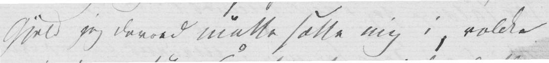Gjeld jeg derved måtte sætte mig i, voldte
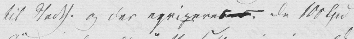til Stockh. og der eqriperede de 100 Spd
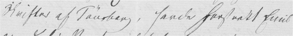Skrifter af Tønsberg, havde forstrakt Emil
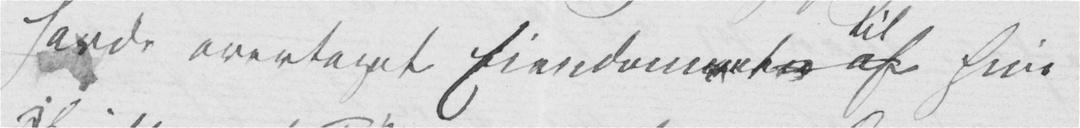havde overtaget Eiendommen af sine
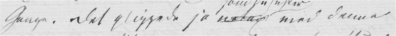Gange. Det glippede jo netop med denne
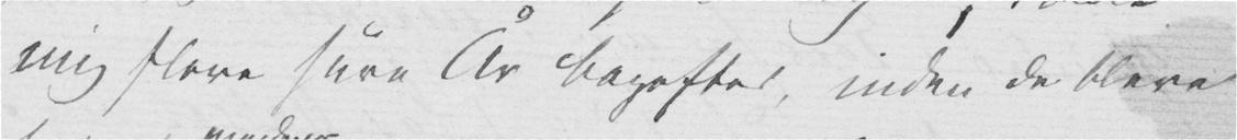mig flere såre År bagefter, inden de bleve
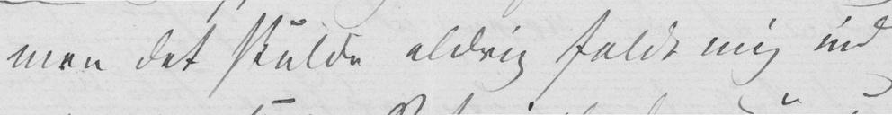men det skulde aldrig faldt mig ind
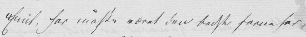Emil, har måske været den bedst farne her,
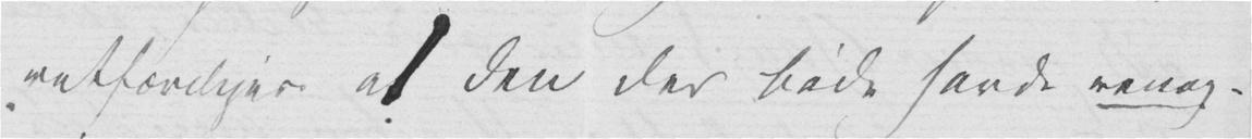retfærdigere at den der både havde renog-
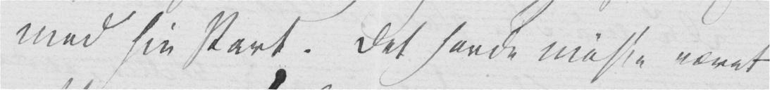med sin Part. Det havde måske været
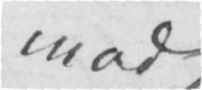mod
